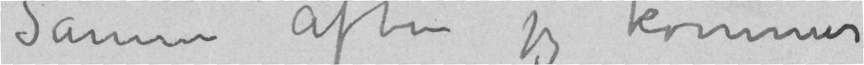Samme Aften jeg kommer
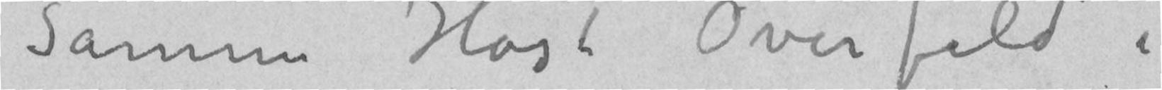Samme Høst Overfald i
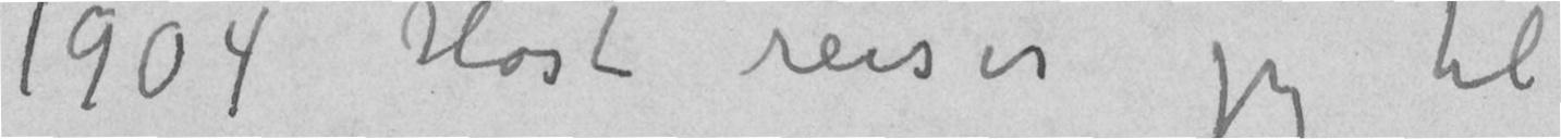1904	Høst reiser jeg til
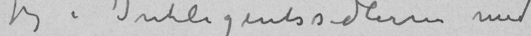jeg i Inteligentssedleren med en
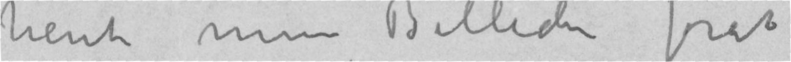hente mine Billeder forat
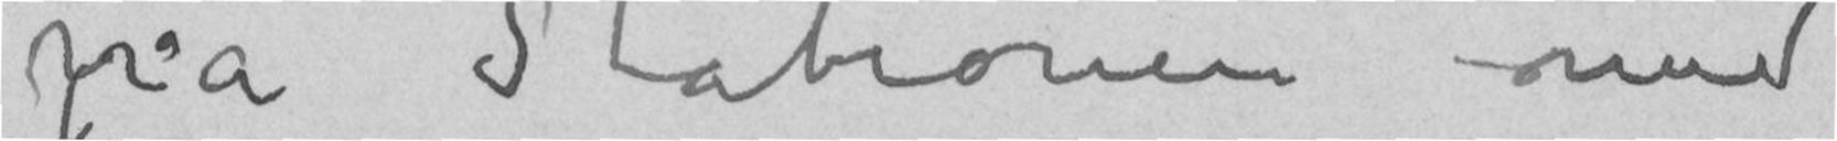på Stationen med
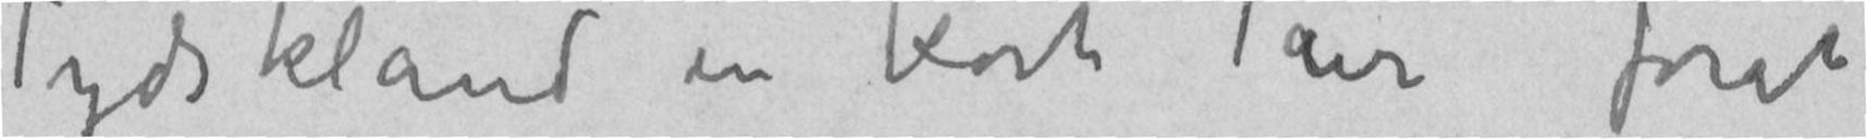Tydskland en kort Tur forat
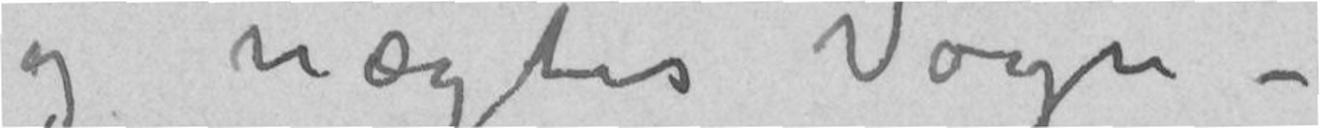og nægtes Vogn –
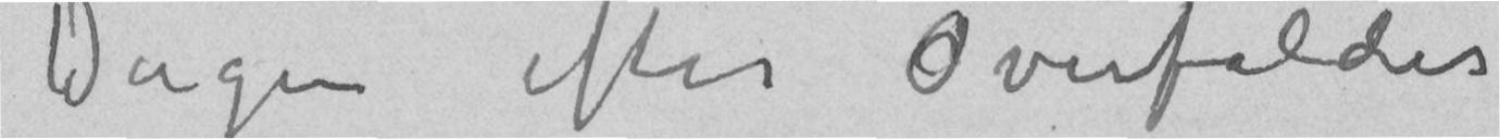Dagen efter overfaldes
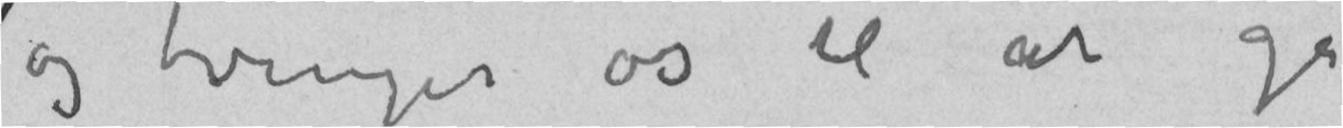og tvinger os til at gå
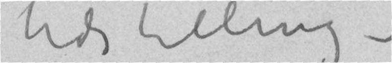Udstilling –
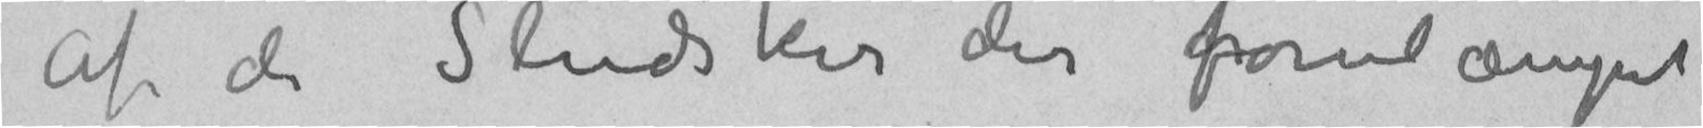Af de Sludsker der forulæmpet
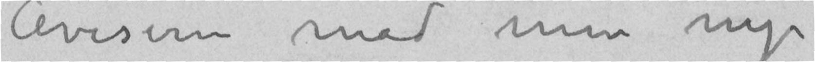Aviserne med min nye
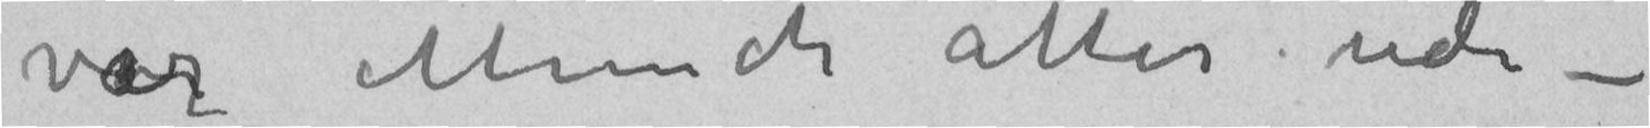var Munch atter ude –
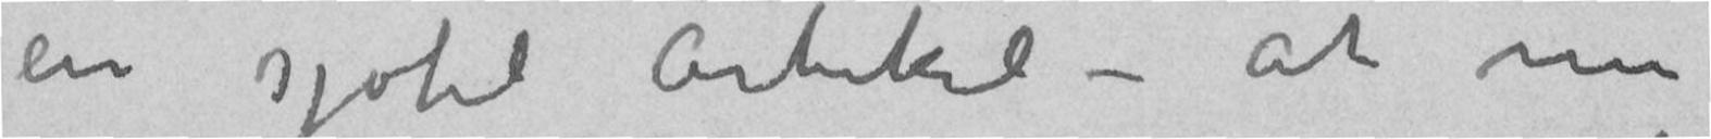sjofel artikkel – at nu
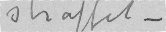straffet –
